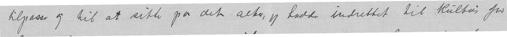tilpasse sig til at sitte paa det alter, jeg hadde indrettet til kultus for
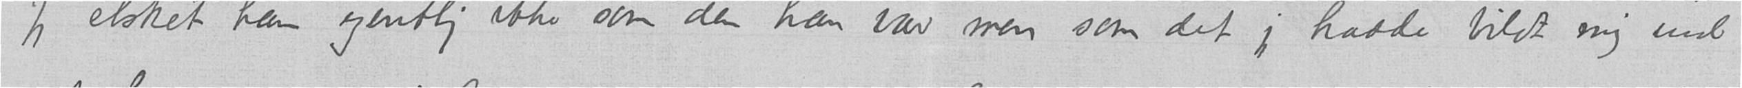jeg elsket ham egentlig ikke som den han var men som det jeg hadde bildt mig ind
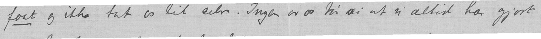faat og ikke tat os til selv. Ingen av os tør si at vi altid har gjort
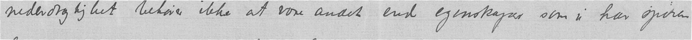nederdrægtighet behøver ikke at være andet end egenskaper som vi har spiren
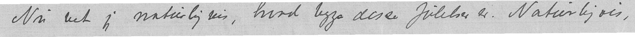Nu vet jeg naturligvis, hvad begge disse følelser er. Naturligvis,
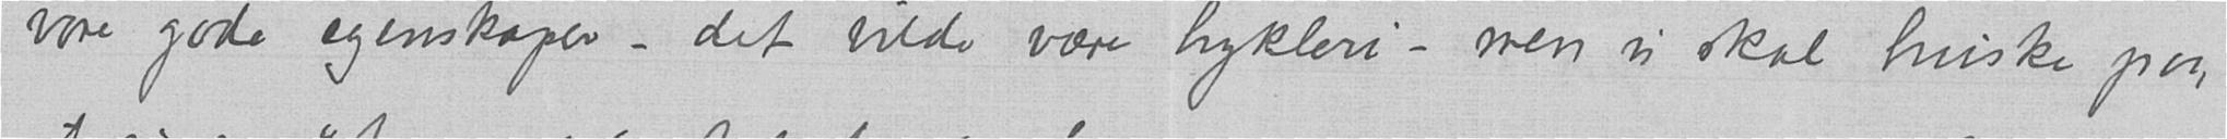vore gode egenskaper – det vilde være hykleri – men vi skal huske paa,
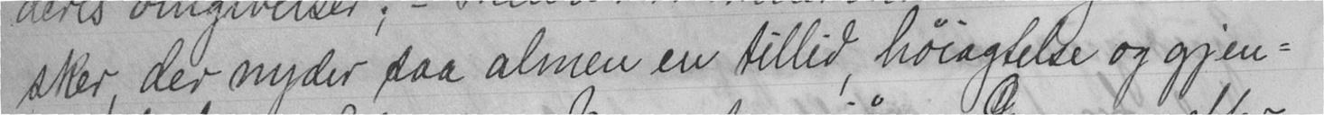sker, der nyder saa almen en tillid, høiagtelse og gjen-
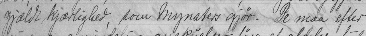gjældt kjærlighed, som Mynsters gjør. De maa efter
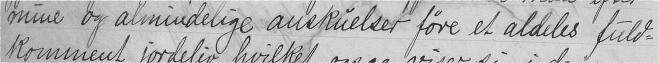mine og almindelige anskuelser føre et aldeles fuld-
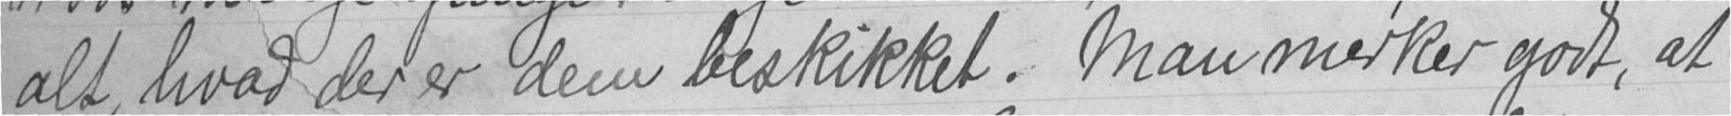alt, hvad der er dem beskikket. Man merker godt, at
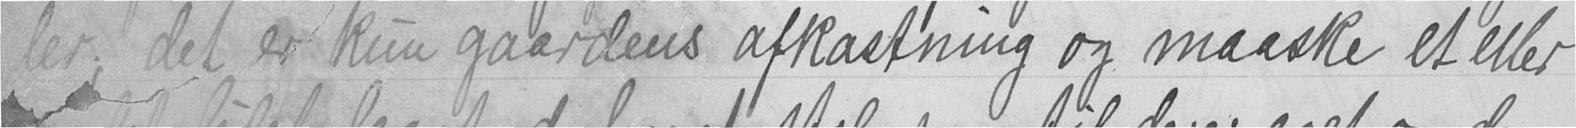ler; det er kun gaardens afkastning og maaske et eller
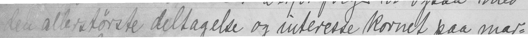den allerstørste deltagelse og interesse kornet paa mar-
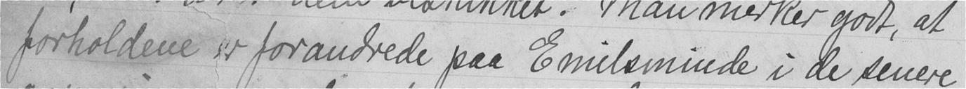forholdene er forandrede paa Emilsminde i de senere
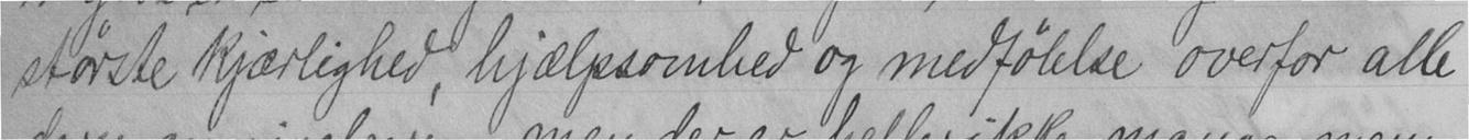største kjærlighed, hjælpsomhed og medfølelse overfor alle
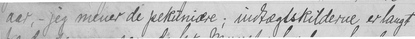aar, - jeg mener de pekuniære; indtægtskilderne er langt
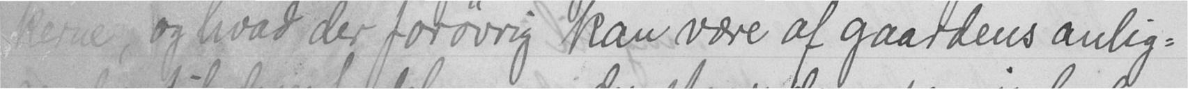kerne, og hvad der forøvrig kan være af gaardens anlig-
